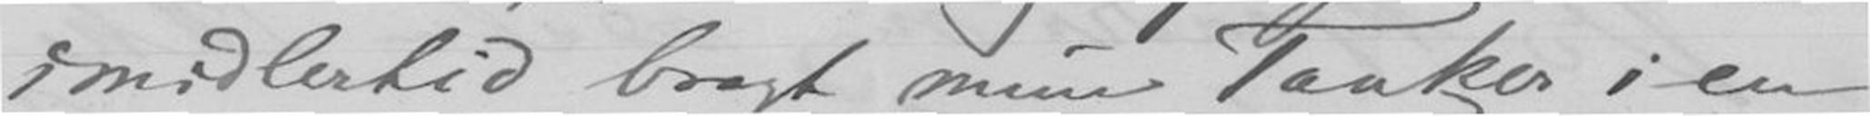imidlertid bragt mine Tanker i en
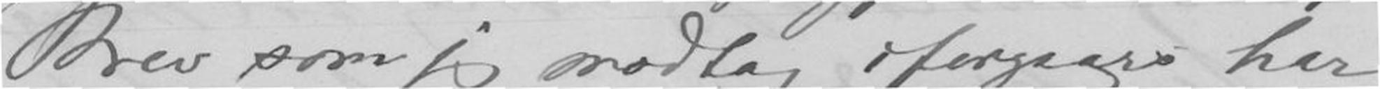Brev som jeg modtog iforgaars har
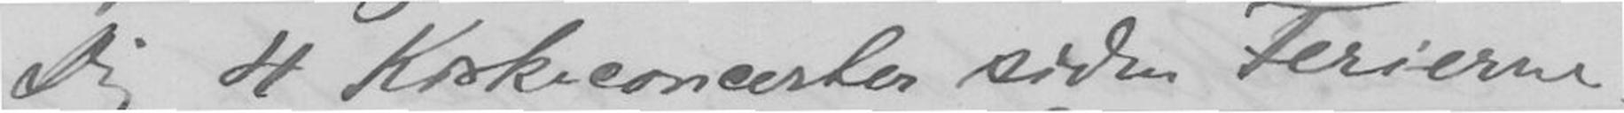Dig 4 Kirkekoncerter siden Ferierne.
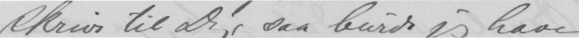skrive til Dig, saa burde jeg have
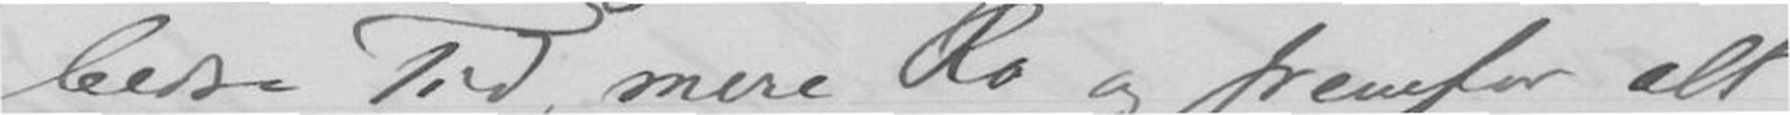bedre Tid, mere Ro og fremfor alt
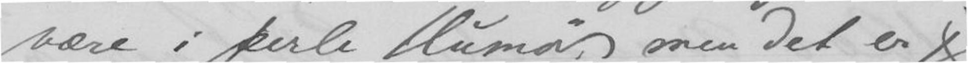være i perle Humør men det er jeg
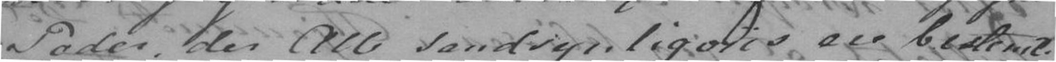Poder, der Alle sandsynligviis ere bestemt
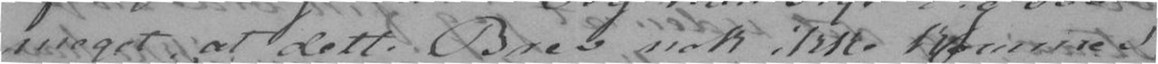meget at dette Brev nok ikke kommer
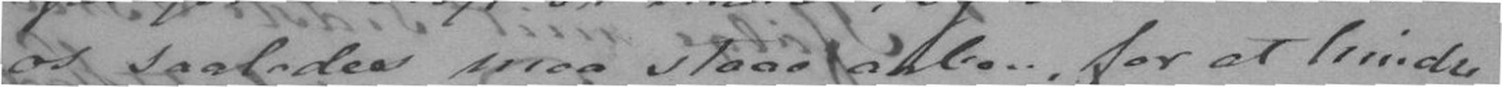os saaledes maa staae aabne, for at hindre
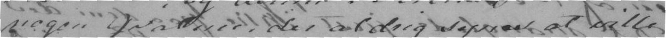nogen Qvalme der aldrig synes at ville
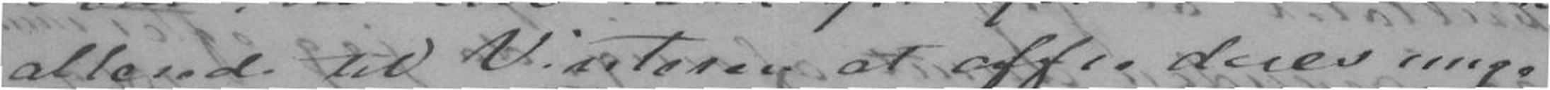allerede til Vinteren at offre deres unge
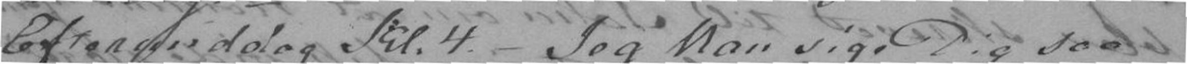Eftermiddag Kl.4. - Jeg kan sige Dig saa
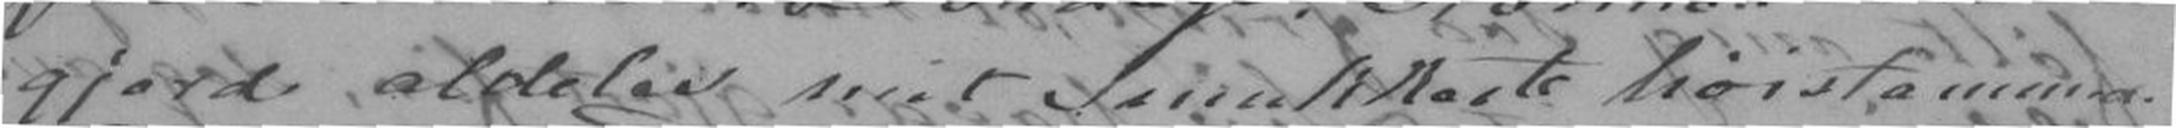gjorde aldeles mit Smukkeste høistammede
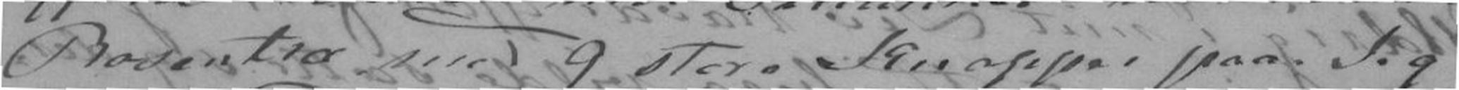Rosentræ med 9 store Knopper paa, Jeg
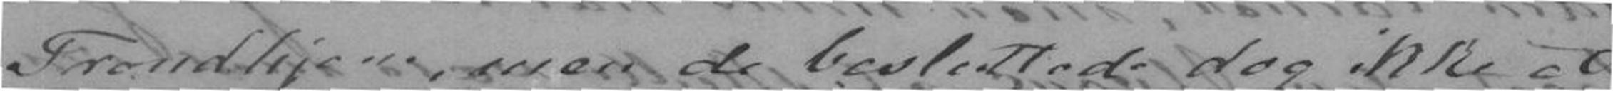Trondhjem, men de besluttede dog ikke at
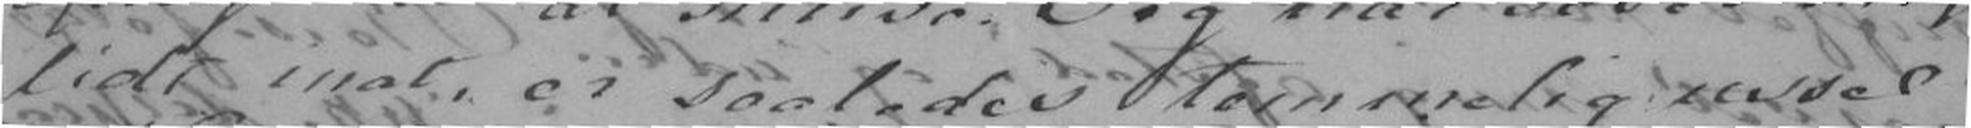lidt inat, er saaledes temmelig ussel
